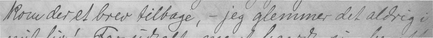kom der et brev tilbage, - jeg glemmer det aldrig i
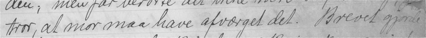tror, at mor maa have afværget det. Brevet gjorde
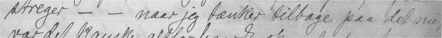streger - - naar jeg tænker tilbage paa det nu,
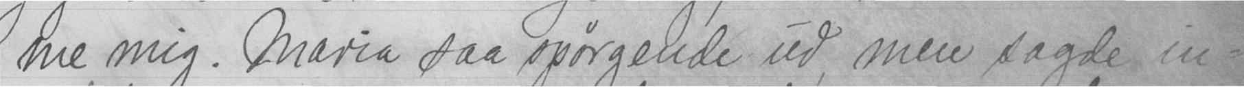me mig. Maria saa spørgende ud, men sagde in-
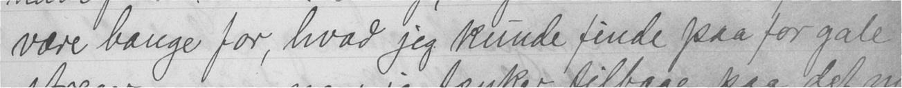være bange for, hvad jeg kunde finde paa for gale
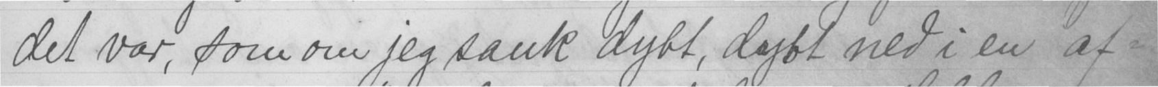det var, som om jeg sank dybt, dybt ned i en af-
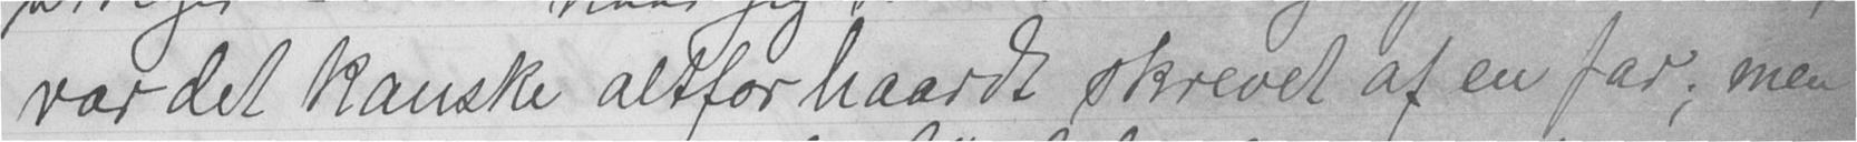var det kanske altfor haardt skrevet af en far; men
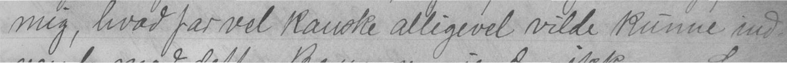mig, hvad far vel kanske alligevel vilde kunne ind-
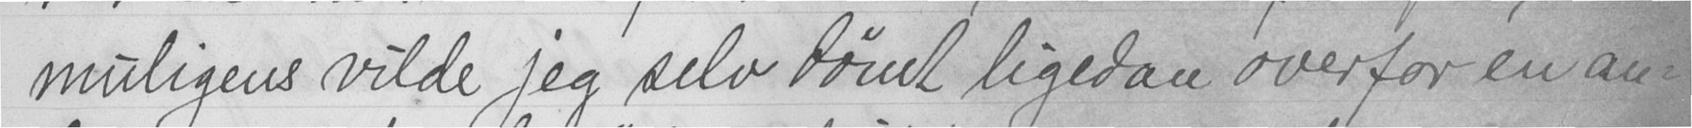muligens vilde jeg selv dømt ligedan overfor en an-
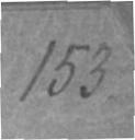153.
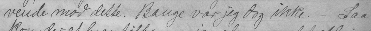vende mod dette. Bange var jeg dog ikke. - Saa
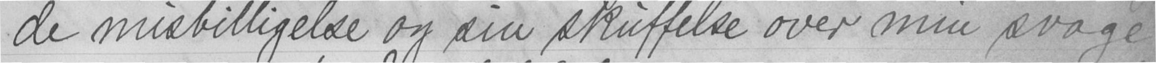de misbilligelse og sin skuffelse over min svage
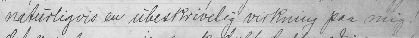naturligvis en ubeskrivelig virkning paa mig!
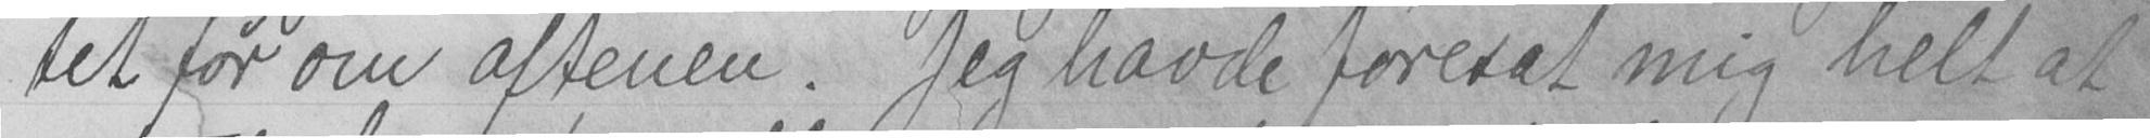tet før om aftenen. Jeg havde foresat mig helt at
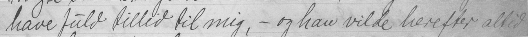have fuld tillid til mig, - og han vilde herefter altid
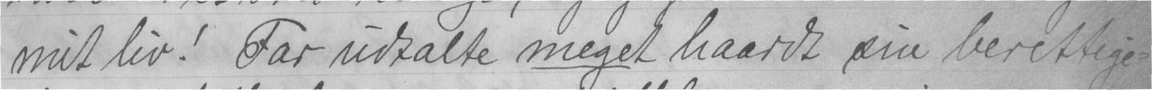mit liv! Far udtalte meget haardt sin berettige-
